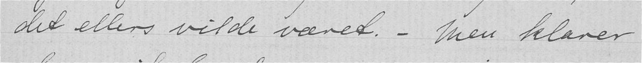det ellers vilde været. - Men klarer
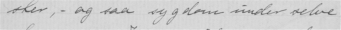ster, - og saa sygdom under selve
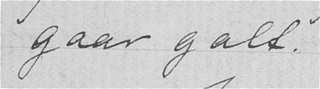gaar galt.
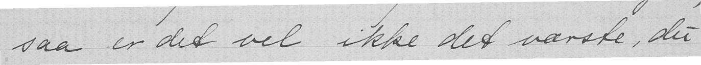saa er det vel ikke det værste, du
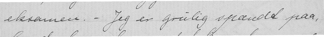eksamen. - Jeg er grulig spændt paa,
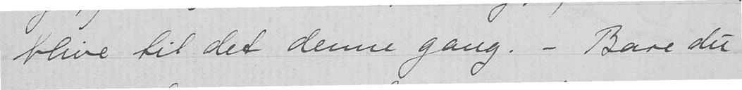blive til det denne gang. - Bare du
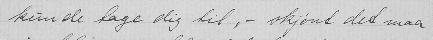kunde tage dig til, - skjønt det maa
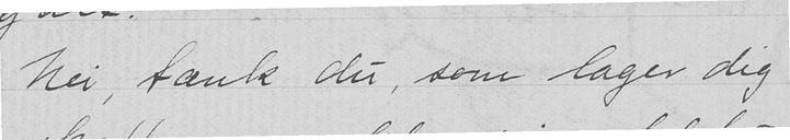Nei, tænk du, som lager dig
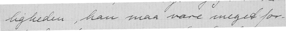ligheden, han maa være meget for-
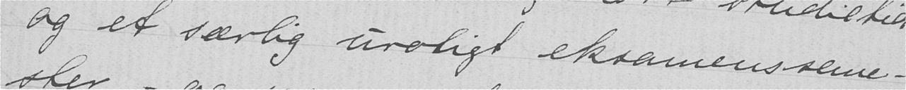og et særlig uroligt eksamensseme-
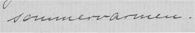sommervarmen.
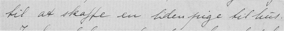til at skaffe en liden pige til hus!
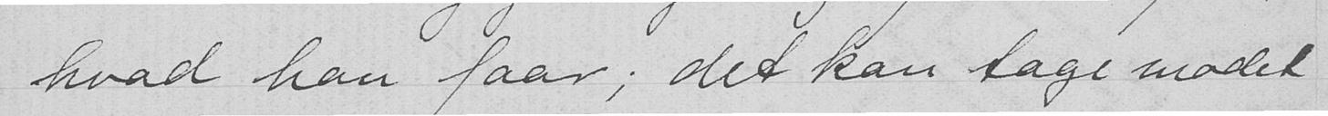hvad han faar; det kan tage modet
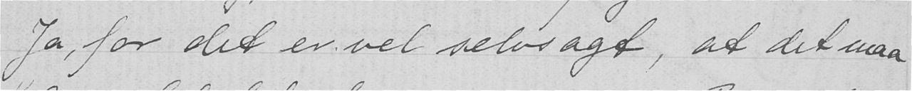- Ja, for det er vel selvsagt, at det maa
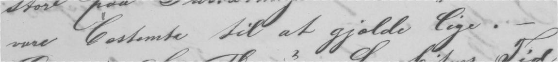vare bestemte til at gjælde lige. -
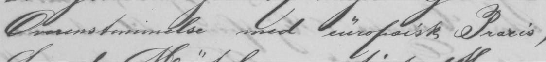Overenstemmelse med europæisk Praxis,
